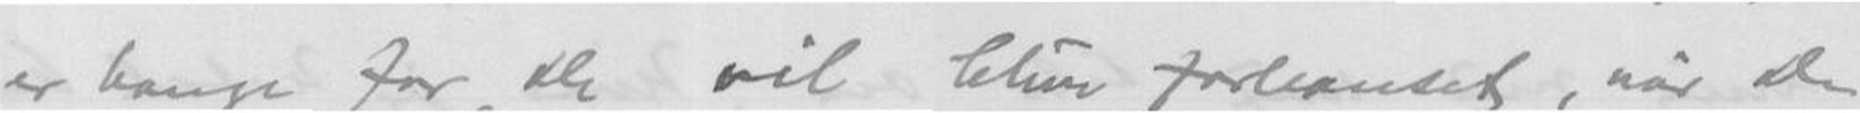er bange for, De vil blive forbandet, når De
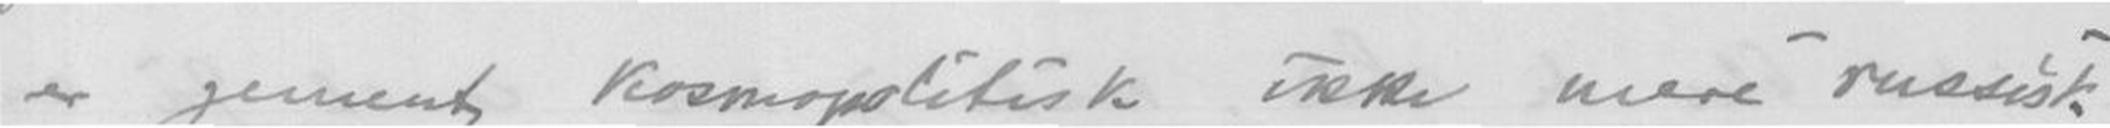er gement kosmopolitisk ikke mere "russisk"
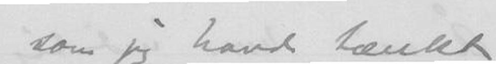som jeg havde tænkt
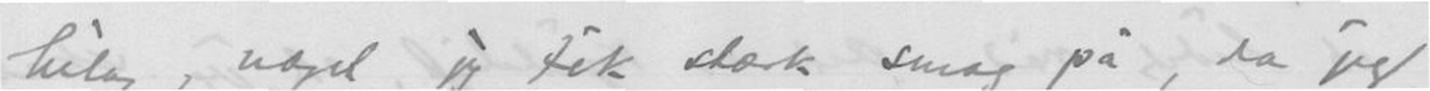bilag, noget jeg fik stærk smag på, da jeg
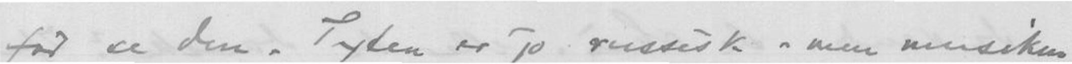får se Dem. Texten er jo russisk, men musiken
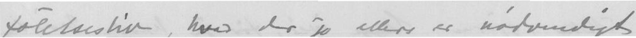følelsesliv, hvad der jo ellers er nødvendigt
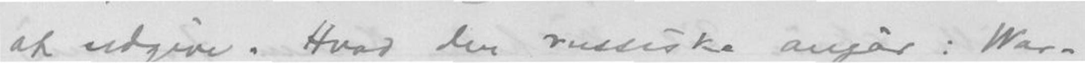at udgive. Hvad den russiske angår: War-
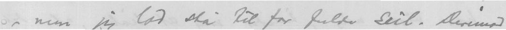men jeg lod stå til for fulde seil. Derimod
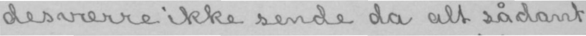desværre ikke sende da alt sådant
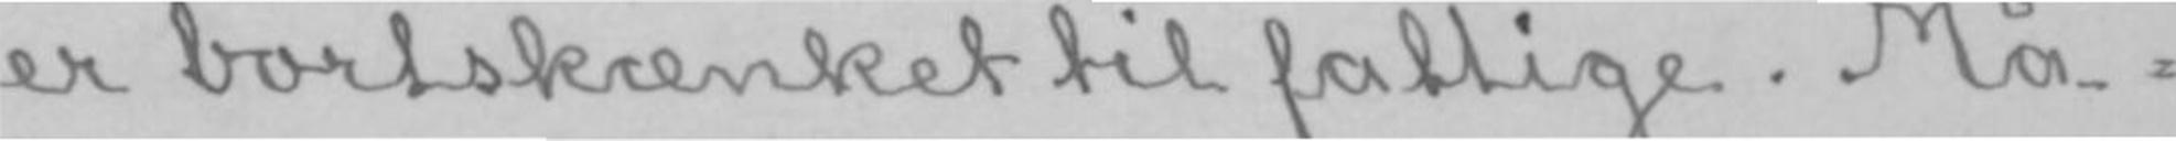er bortskænket til fattige. Må-
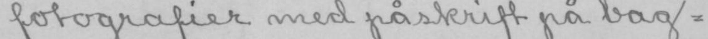fotografier med påskrift på bag-
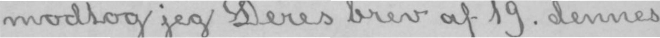modtog jeg Deres brev af 19. dennes,
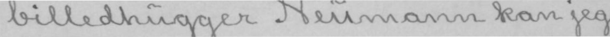billedhugger Neumann kan jeg
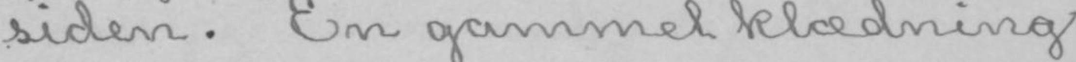siden. En gammel klædning
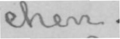chen.
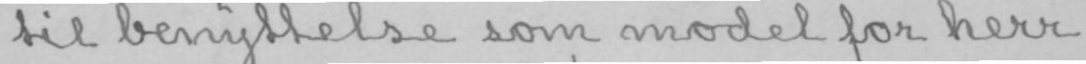til benyttelse som model for herr
